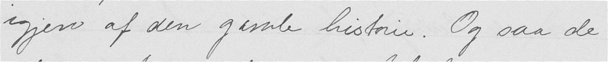igjen af den gamle historie. Og saa de
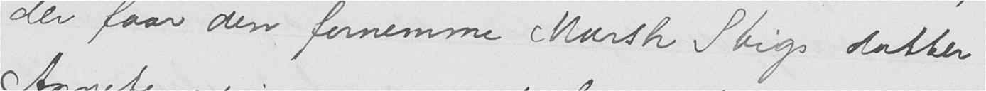der faar den fornemme Marsk Stigs datter
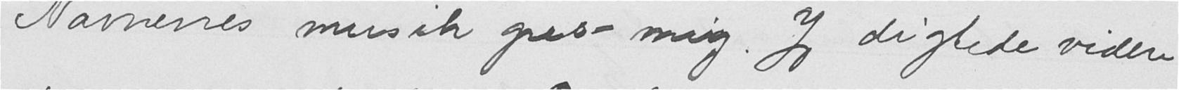Navnenes musik greb mig. Jeg digtede videre
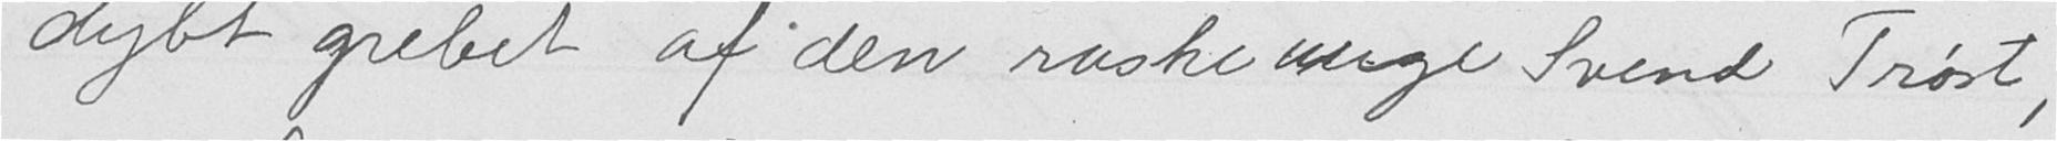dybt grebet af den raske unge Svend Trøst,
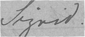Sigrid
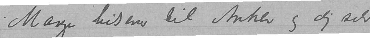Mange hilsener til Anker og dig selv
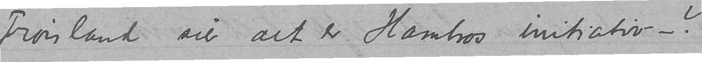Frøisland sier det er Hambros initiativ –-?
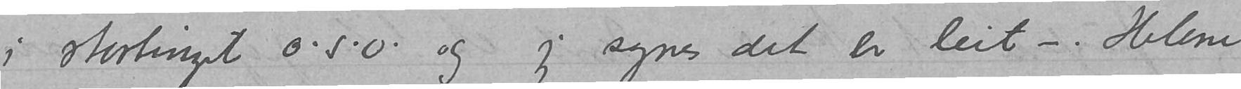i stortinget o.s.v. og jeg synes det er leit –-.  Helene
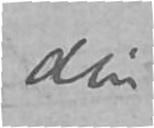din
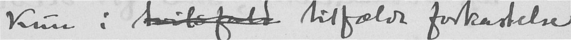Kun i tvilsfald tilfælde forkastelse
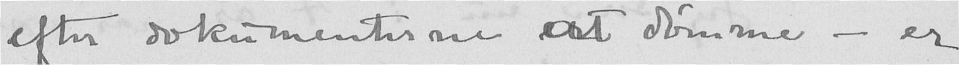efter dokumenterne at dømme - er
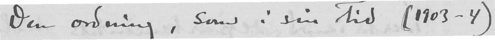Den ordning, som i sin tid (1903-4)
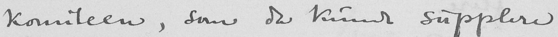komiteen, som da kunde supplere
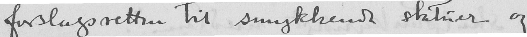forslagsretten til smykkende statuer og
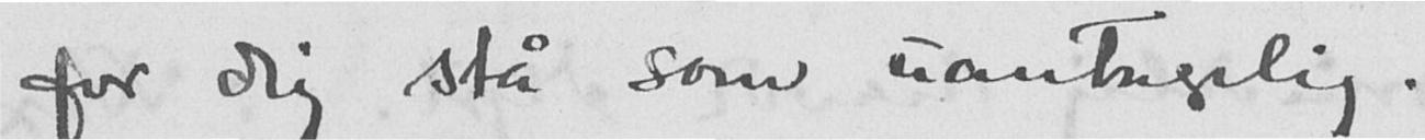for dig stå som uantagelig.
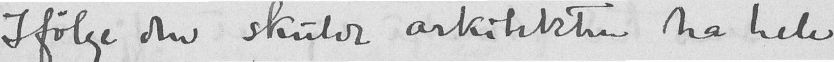Ifølge den skulde arkitekten ha hele
